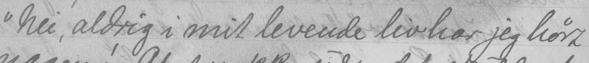"Nei, aldrig i mit levende liv har jeg hørt
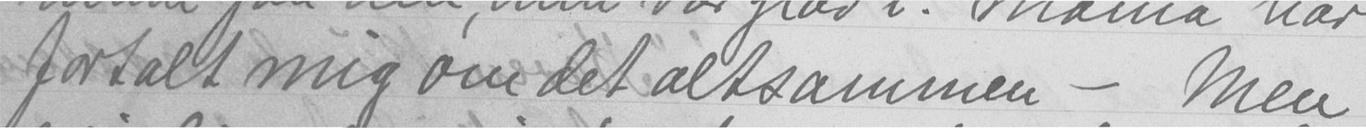fortalt mig om det altsammen - Men
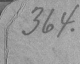364.
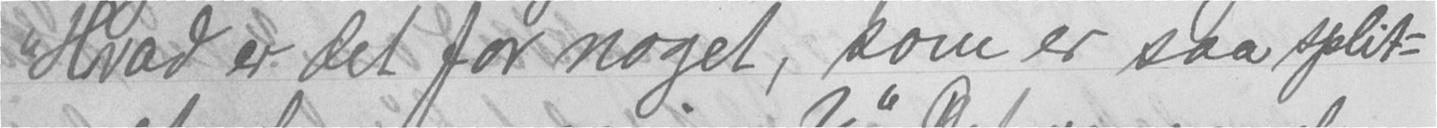"Hvad er det for noget, som er saa split-
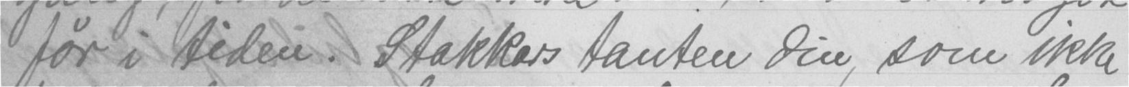før i tiden. Stakkars tanten din, som ikke
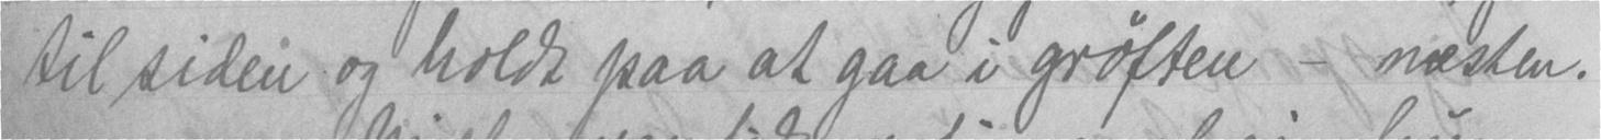til siden og holdt paa at gaa i grøften - næsten.
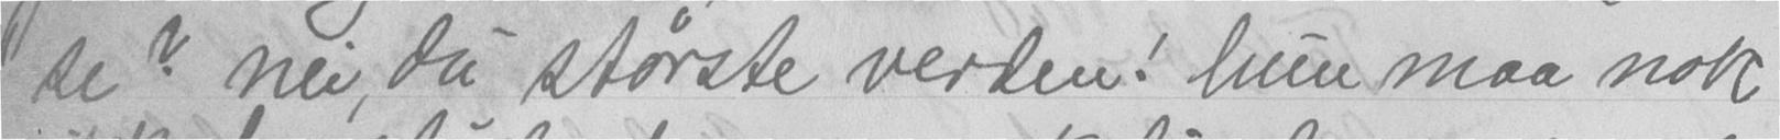se? nei, du største verden! hun maa nok
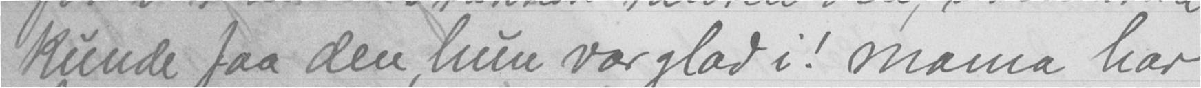kunde faa den, hun var glad i! mama har
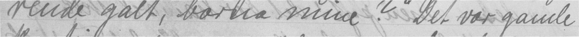rende galt, barna mine?" Det var gamle
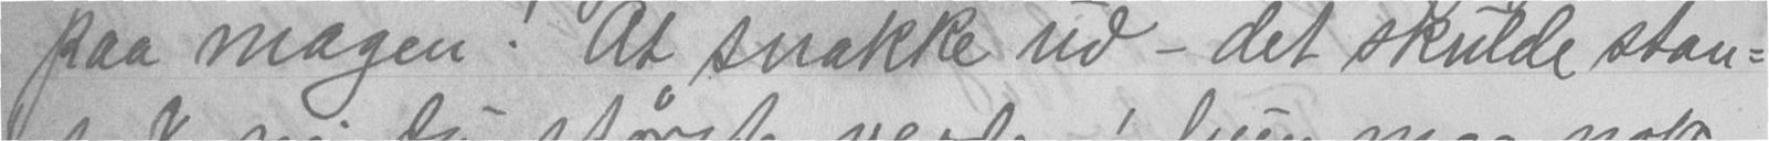paa magen! At snakke ud - det skulde stan-
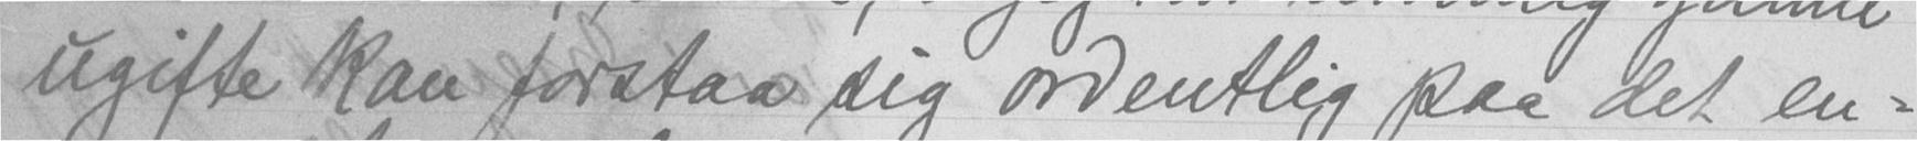ugifte kan forstaa sig ordentlig paa det en-
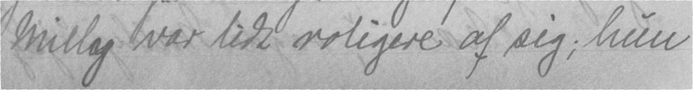Milly var lidt roligere af sig; hun
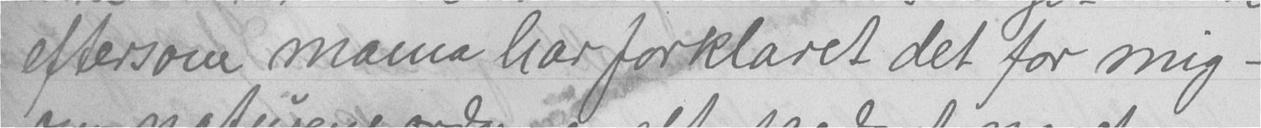eftersom mama har forklaret det for mig -
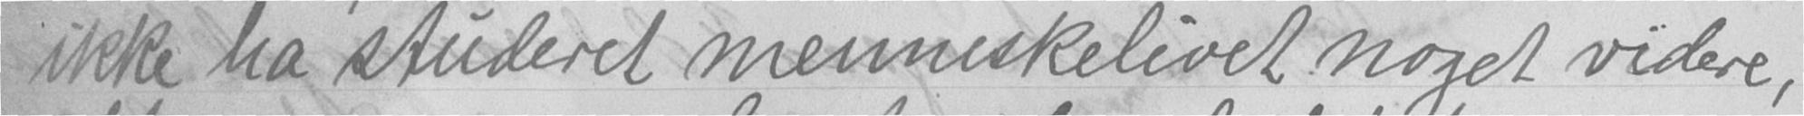ikke ha studeret menneskelivet noget videre,
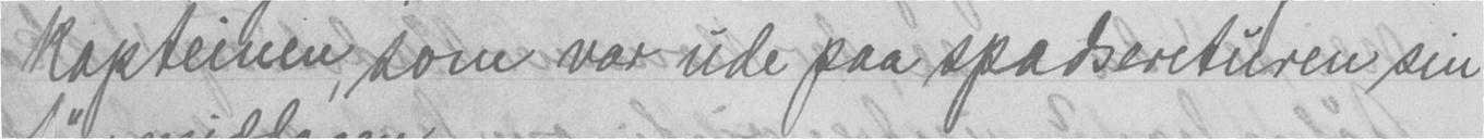kapteinen, som var ude paa spadsereturen sin
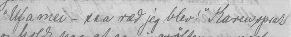"Uf a mei - saa ræd jeg blev!" Karen sprat
